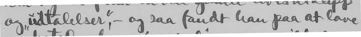og "udtalelser", - og saa fandt han paa at lave
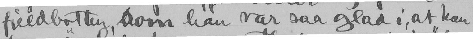fjeldbotten, som han var saa glad i, at han
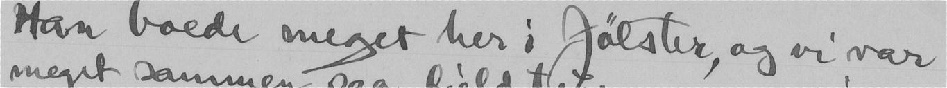Han boede meget her i Jølster, og vi var
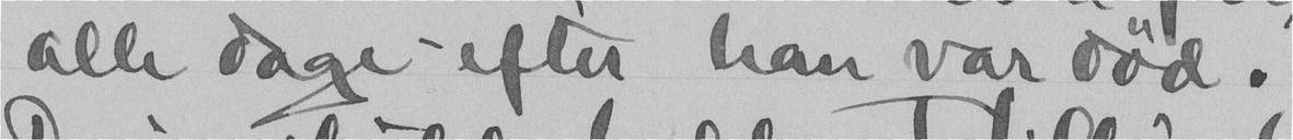alle dage - efter han var død.
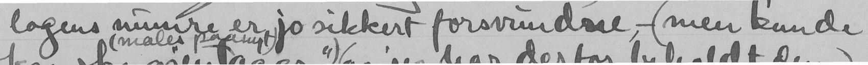logens numre er jo sikkert forsvundne - (men kunde
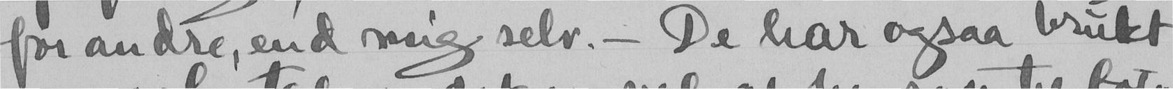for andre, end mig selv. - De har ogsaa brukt
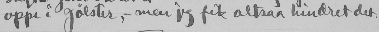oppe i Jølster, - men jeg fik altsaa hindret det
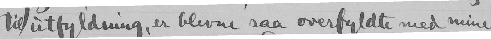til utfyldning, er blevne saa overfyldte med mine
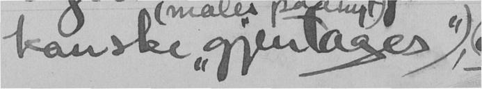kanske "gjentages").
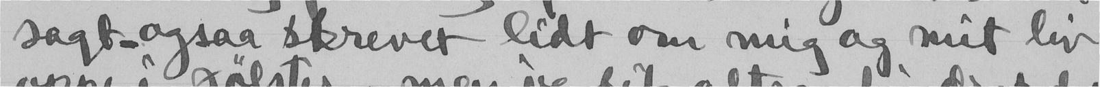sagt, ogsaa skrevet lidt om mig og mit liv
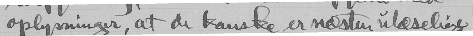oplysninger, at de kanske er næsten ulæselige
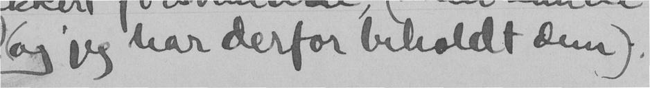(og jeg har derfor beholdt dem).
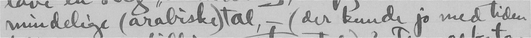mindelige (arabiske) tal, - (der kunde jo med tiden
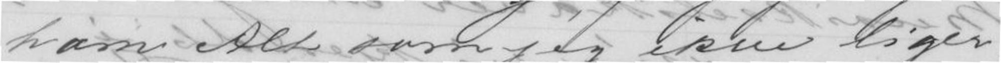ham Alt som jeg ikke liger
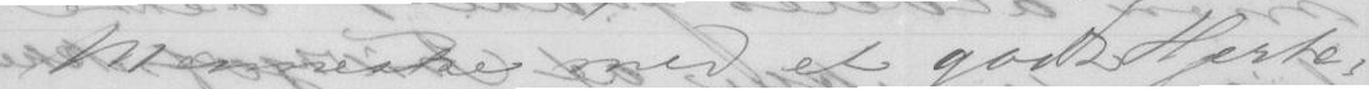Menneske med et godt Hjerte-
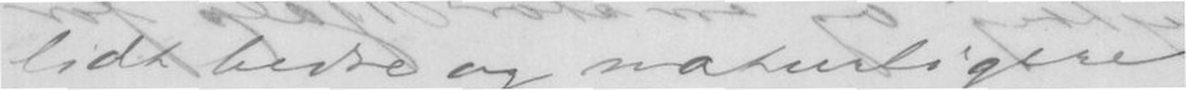lidt bedre og naturligere
